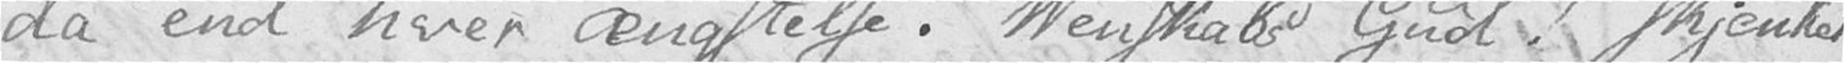da end hver ængstelse. Venskabs Gud! skjenker
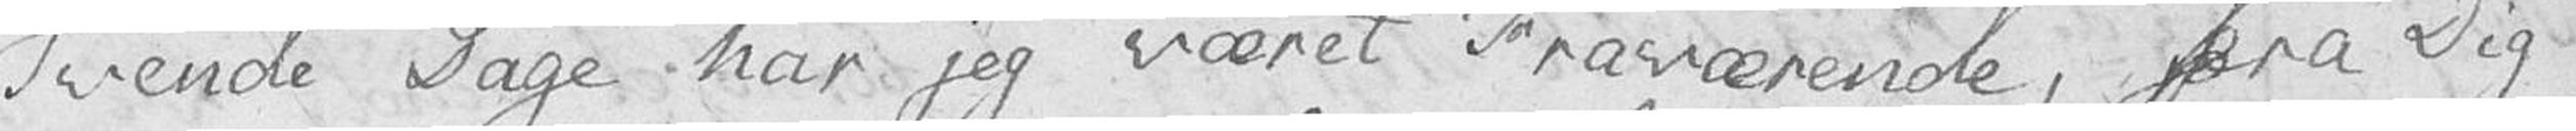Tvende Dage har jeg været Fraværende, fra Dig
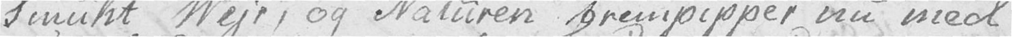Smukt Vejr, og Naturen frempipper nu med
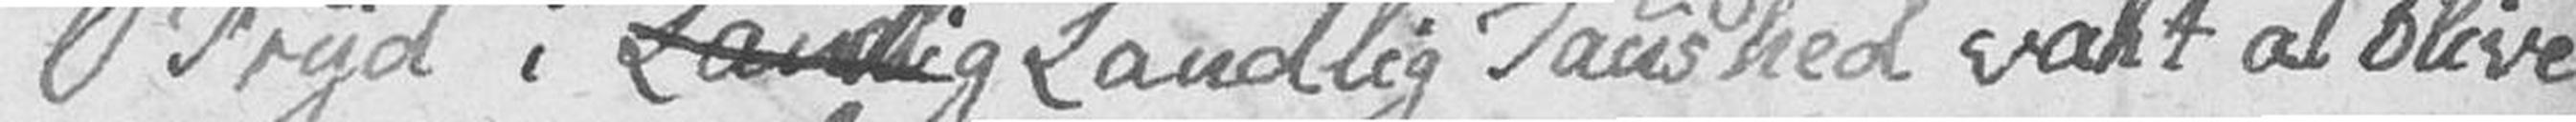O Fryd i Landlig Landlig Taushed vakt at blive
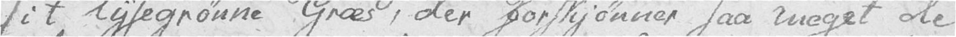sit lysegrønne Græs, der forskjønner saa meget de
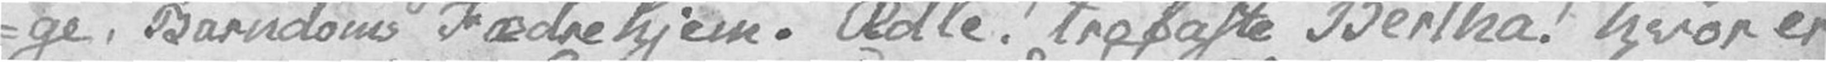-ge, Barndoms Fædrehjem. Ædle! trofaste Bertha! hvor er
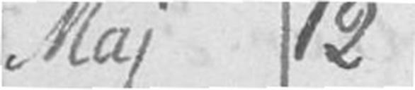Maj 12
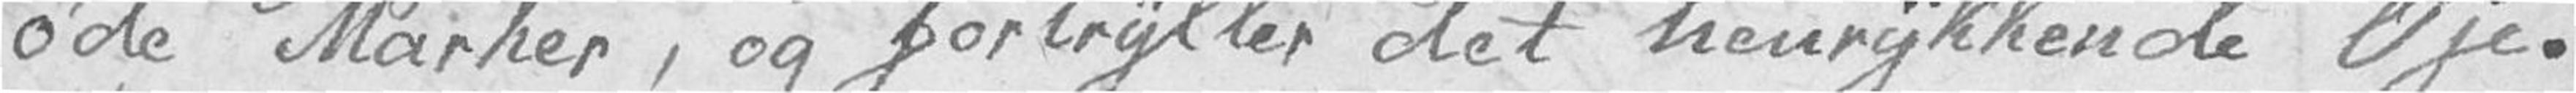øde Marker, og fortryller det henrykkende Øje.
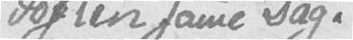Aften samme Dag.
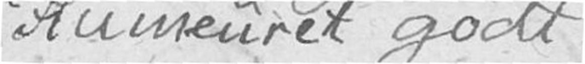Humeuret godt
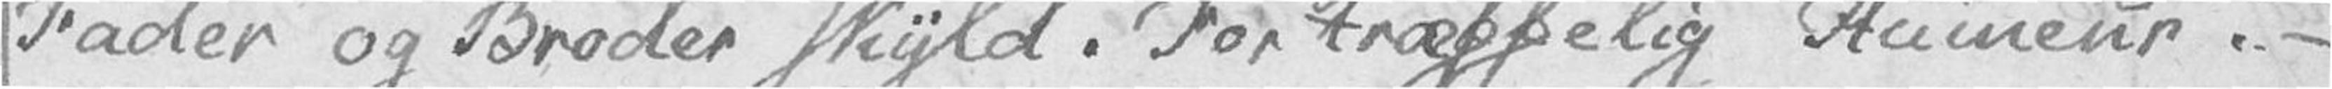Fader og Broder skyld. Fortræffelig Humeur. –
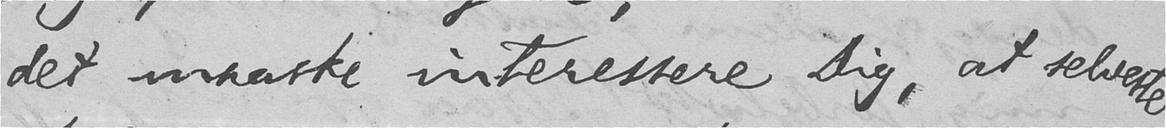det maaske interessere Dig, at selveste
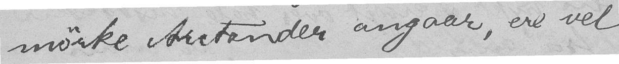mørke Arctander angaar, ere vel
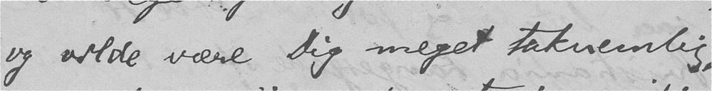og vilde være Dig meget taknemlig,
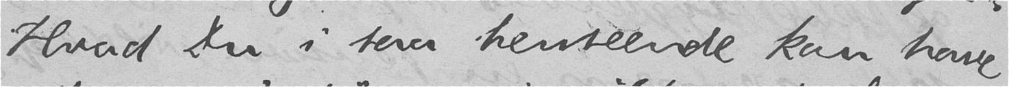Hvad Du i saa henseende kan have
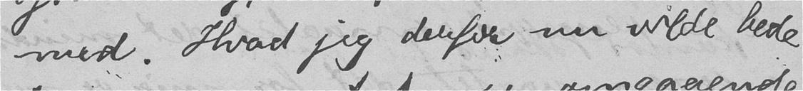med. Hvad jeg derfor nu vilde bede
